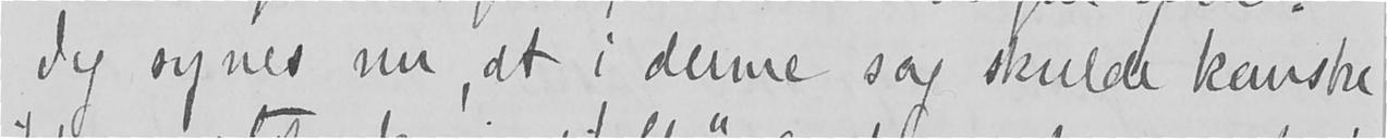Jeg synes nu at i denne sag skulde kanske
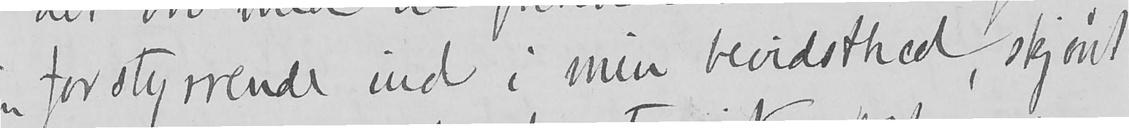forstyrrende ind i min bevidsthed, skjønt
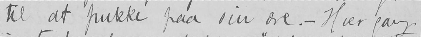til at pukke paa sin ære. - Hver gang
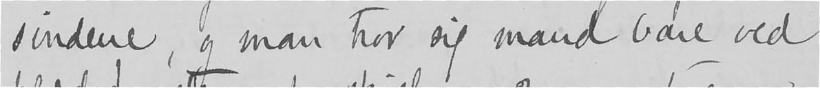sindene, og man tror sig mand bare ved
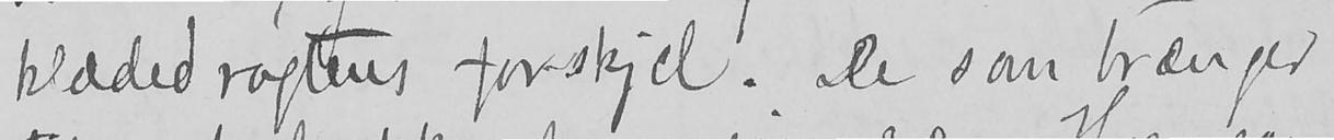klædedragtens forskjel. De som trænger
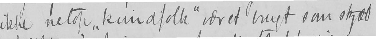ikke netop "kvindfolk" været brugt som skjæl
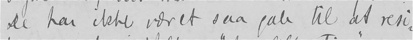De har ikke været saa gale til at resi-
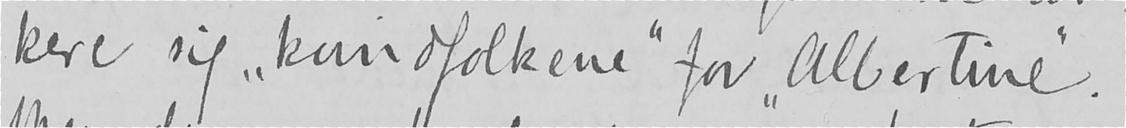kere sig "kvindfolkene" for "Albertine".
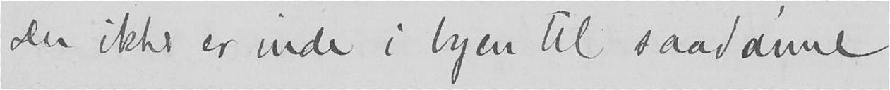Du ikke er inde i byen til saadanne
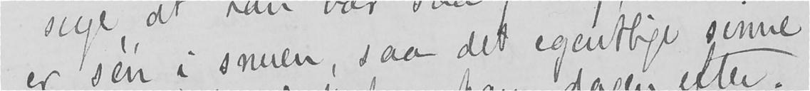er sén i snuen, saa det egentlige sinne
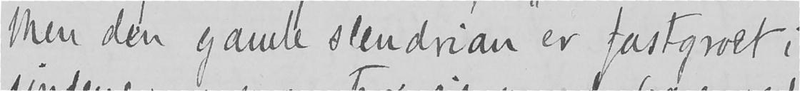Men den gamle slendrian er fastgroet i
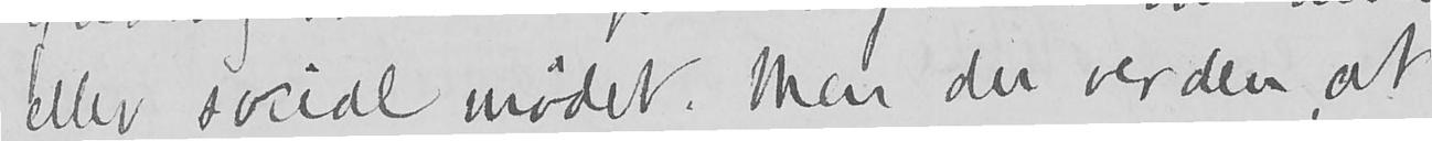eller social mødet. Men du verden, at
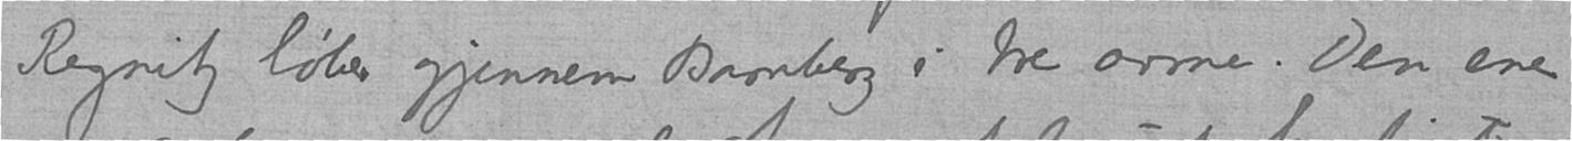Regnitz løber gjennem Bamberg i tre arme. Den ene
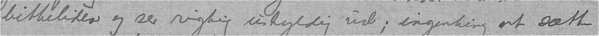bitteliden og ser rigtig uskyldig ud; ingenting at sætte
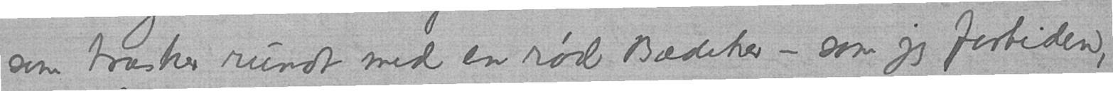som trasker rundt med en rød Bædiker - som jeg fortiden,
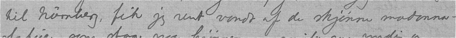til Nürnberg, fik jeg rent vondt af de skjønne madonna-
